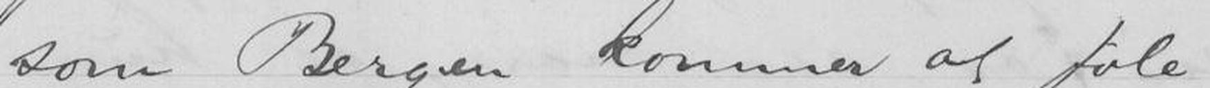som Bergen kommer at føle
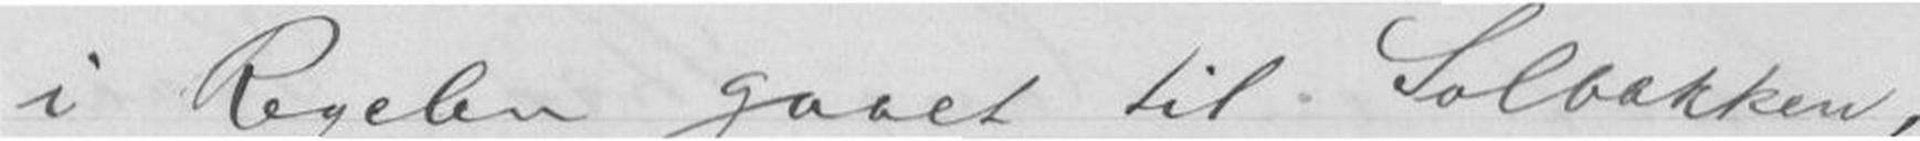i Regelen gaaet til Solbakken,
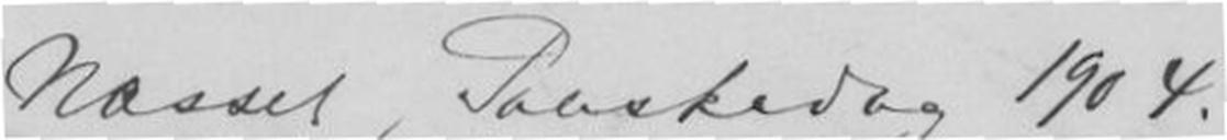Næsset Paaskedag 1904.
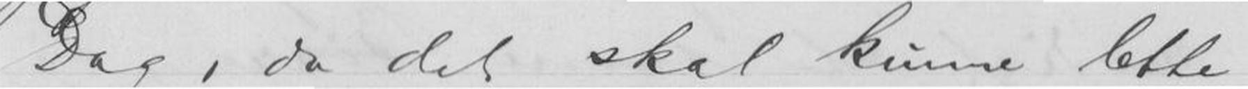Dag, da det skal kunne lette
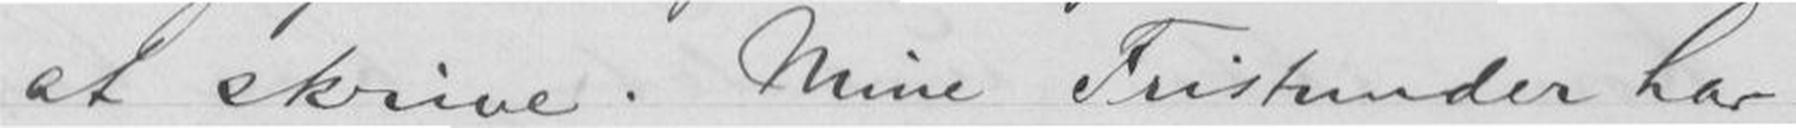at skrive. Mine Fristunder har
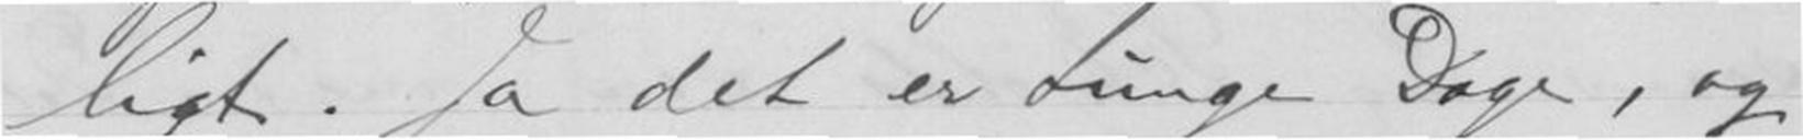ligt. Ja det er tunge Dage, og
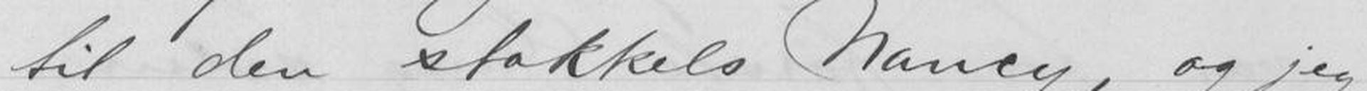til den stakkels Nancy, og jeg
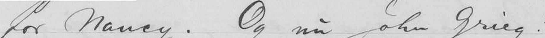for Nancy. Og nu John Grieg!
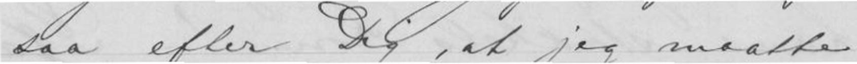saa efter Dig, at jeg maatte
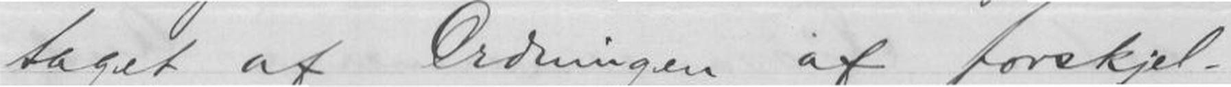taget af Ordningen af forskjel-
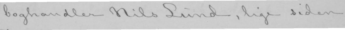boghandler Nils Lund, lige siden
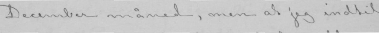December måned, men at jeg indtil
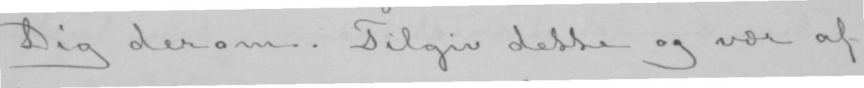Dig derom. Tilgiv dette og vær af
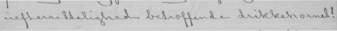uefterrettelighed betræffende drikkehornet!
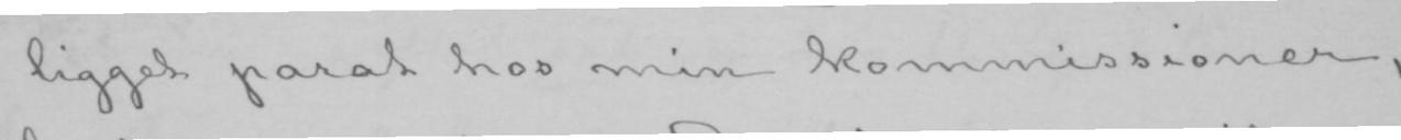ligget parat hos min kommissioner,
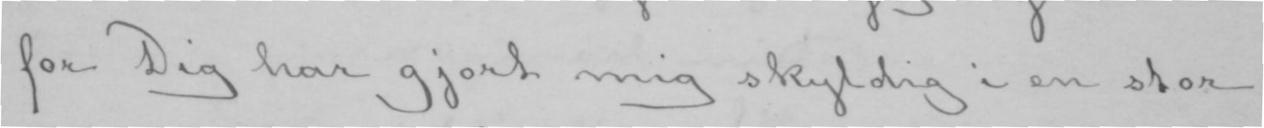for Dig har gjort mig skyldig i en stor
